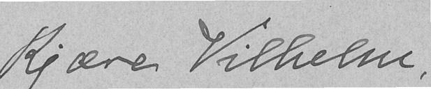Kjære Vilhelm, -
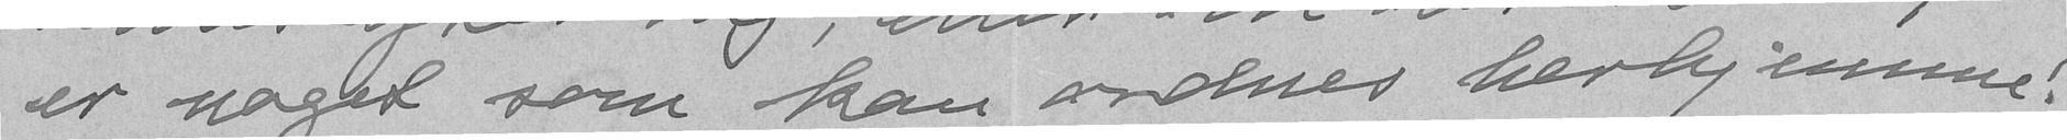er noget som kan ordnes herhjemme!
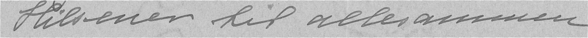Hilsener til allesammen
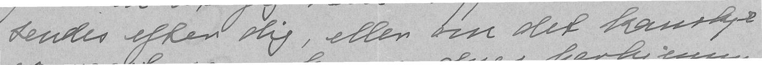sendes efter dig, eller om det kanskje
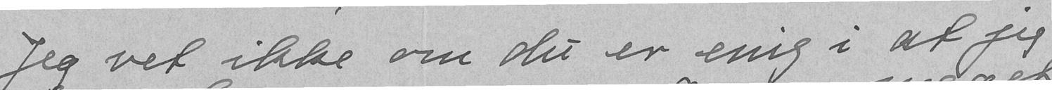Jeg vet ikke om du er enig i at jeg
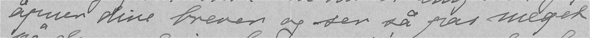åpner dine brever og ser så pas meget
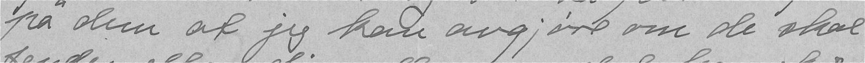på dem at jeg kan avgjøre om de skal
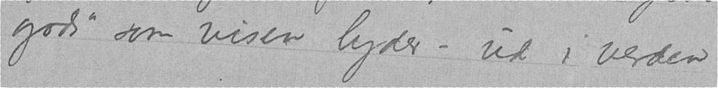gods" som visen lyder - ud i verden
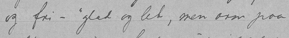og fri - "glad og let, men arm paa
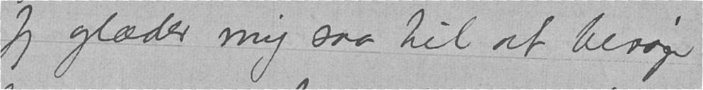Jeg glæder mig saa til at besøge
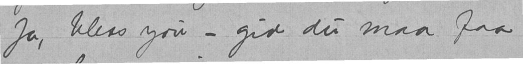Ja, bless you - gid du maa faa
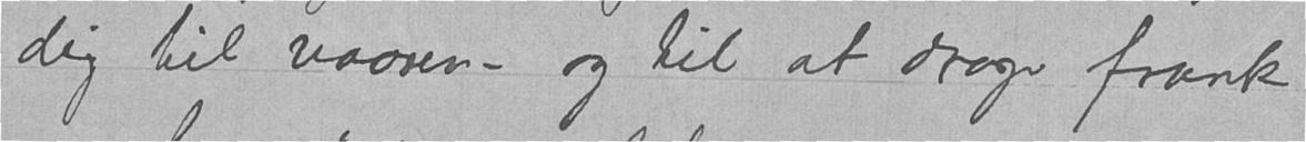dig til vaaren - og til at drage frank
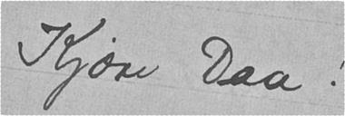Kjære Dea!
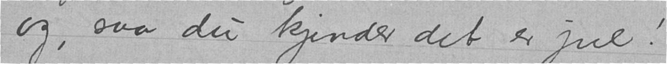og, saa du kjender det er jul!
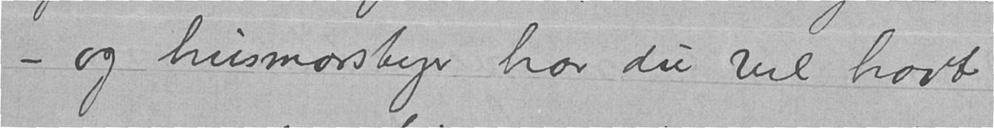- og husmorstyr har du vel havt
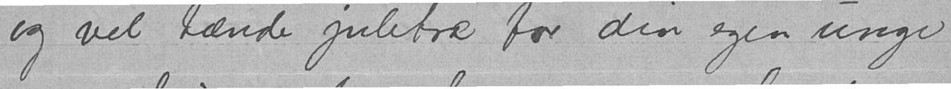og vel tænde juletræ for din egen unge
